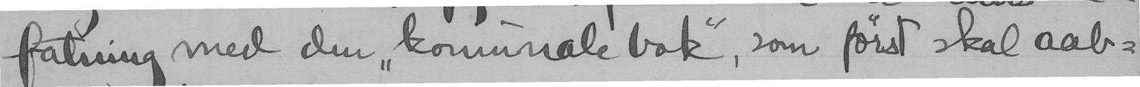fatning med den "komunale bok", som først skal aab-
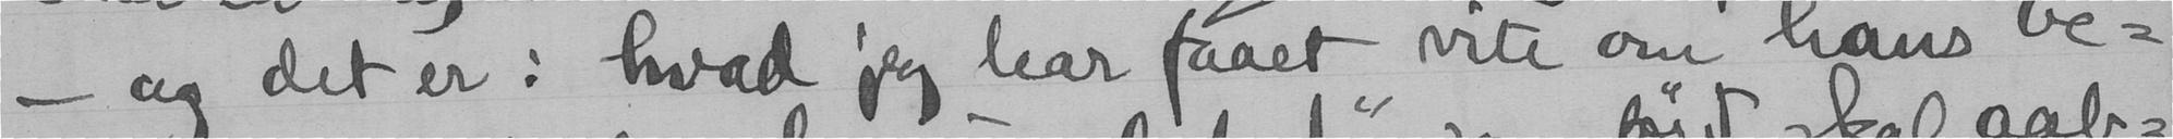- og det er: hvad jeg har faaet vite om hans be-
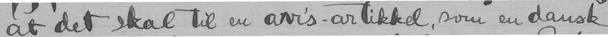at det skal til en avis artikkel, som en dansk
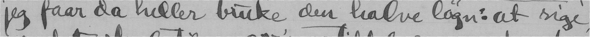jeg faar da heller bruke den halve løgn: at sige,
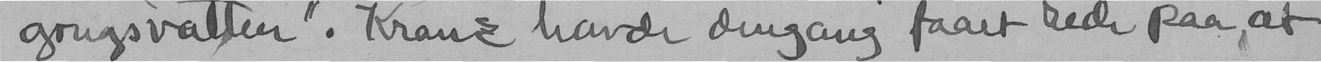gongsvatten". Kranz havde dengang faaet rede paa, at
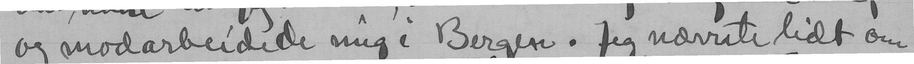os modarbeidede mig i Bergen. Jeg nævnte lidt om
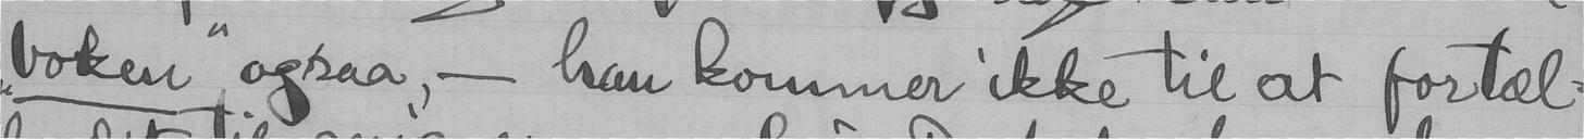"boken" ogsaa, - han kommer ikke til at fortæl-
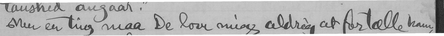Men en ting maa De love mig aldrig at fortælle ham,
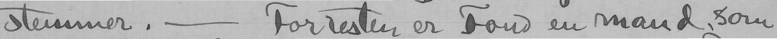stemmer . - Forresten er Fond en mand, som
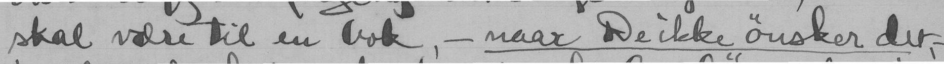skal være til en bok, - naar De ikke ønsker det,-
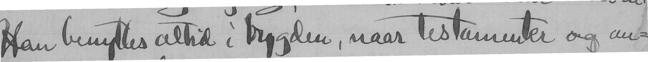Han benyttes altid i bygden, naar testamenter og an-
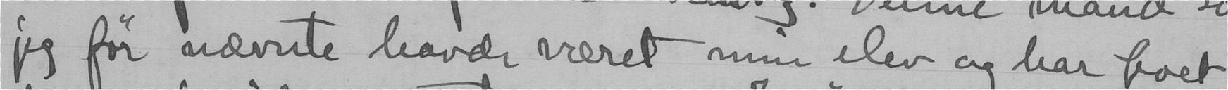jeg før nævnte havde været min elev og har boet
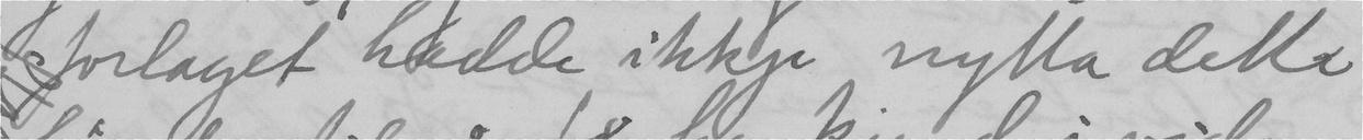forlaget hadde ikkje nytta dette
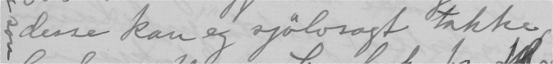desse kan eg sjölvsagt takke
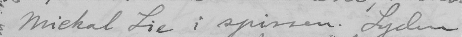Mickal Lie i spissen. Lyden
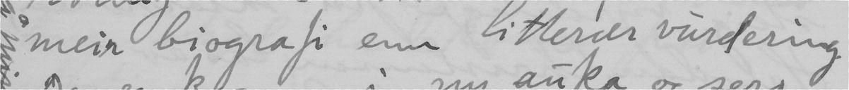meir biografi enn litterær vurdering.
